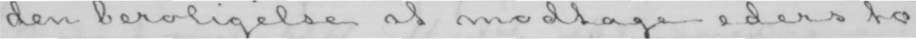den beroligelse at modtage eders to
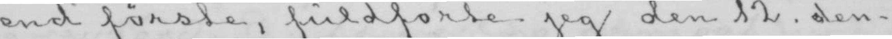end første, fuldførte jeg den 12. den-
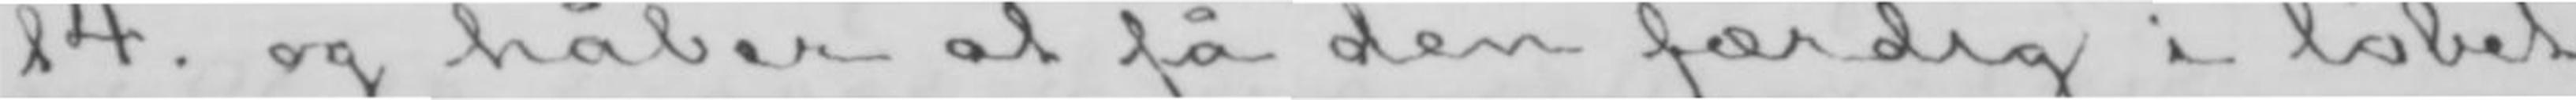14. og håber at få den færdig i løbet
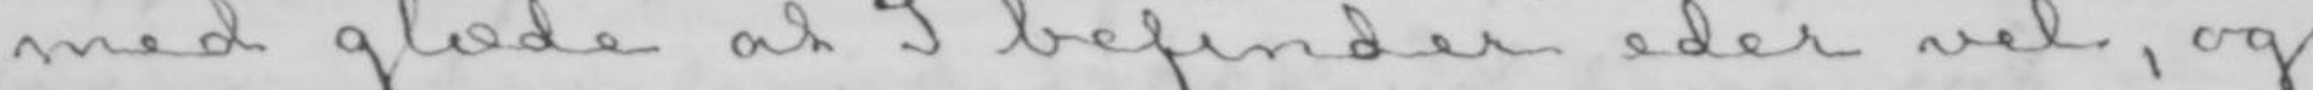med glæde at I befinder eder vel, og
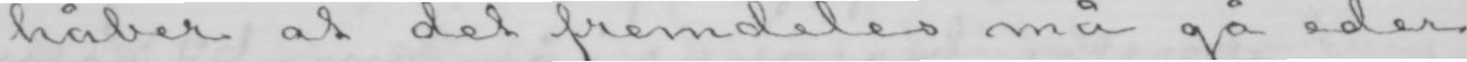håber at det fremdeles må gå eder
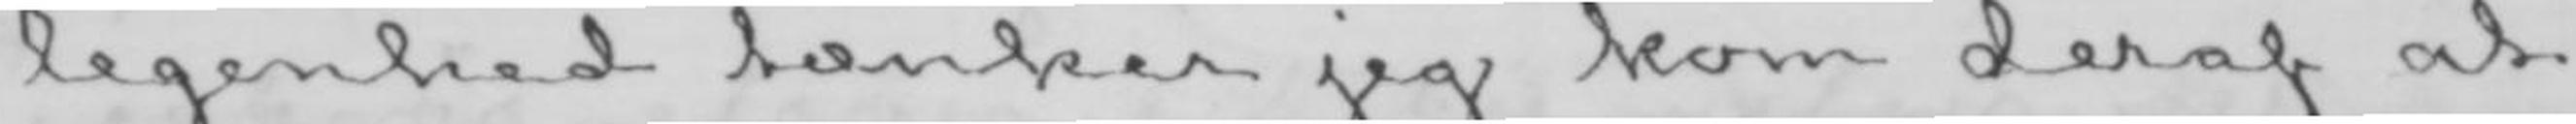legenhed tænker jeg kom deraf at
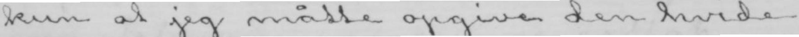kun at jeg måtte opgive den hvide
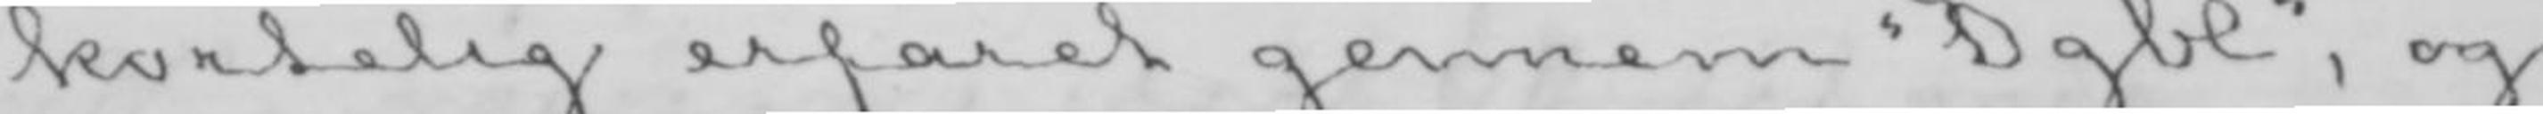jo kortelig erfaret gennem «Dgbl», og
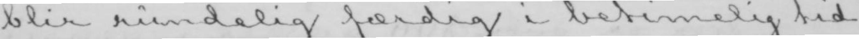blir rundelig færdig i betimelig tid.
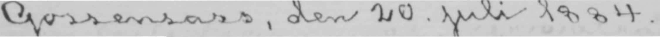Gossensass, den 20. juli 1884.
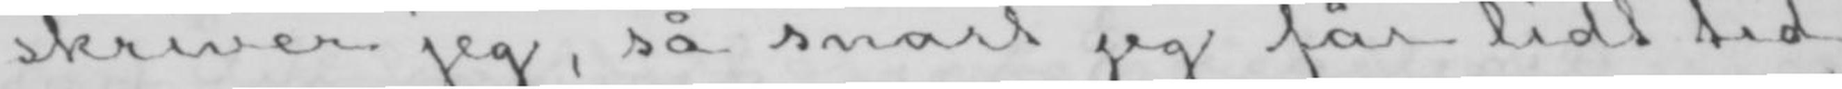skriver jeg, så snart jeg får lidt tid
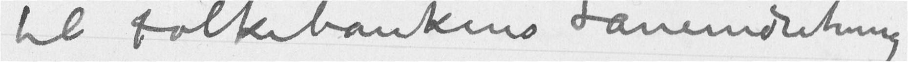til Folkebankens Låneindretning
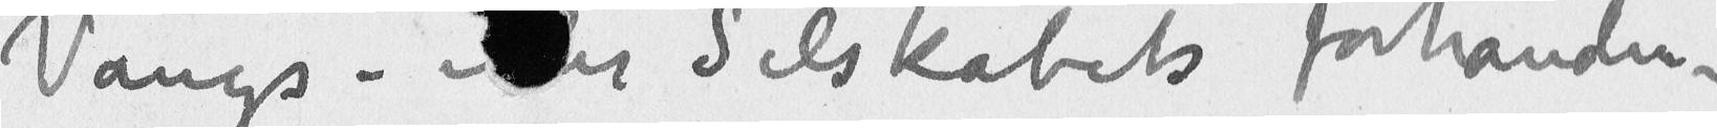Vangs – Selskabets forhånden-
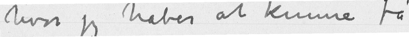hvor jeg håber at kunne få
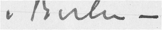i Berlin –
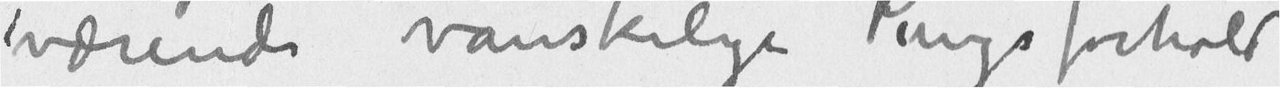værende vanskelige Pengeforhold
